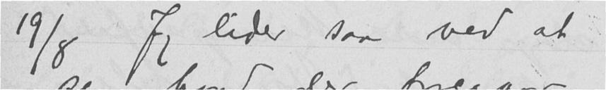19/8 Jeg lider saa ved at
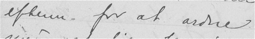efterm. for at ordne
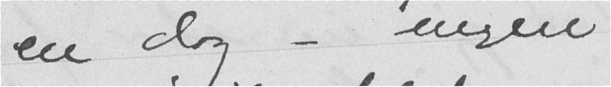en dag - ingen
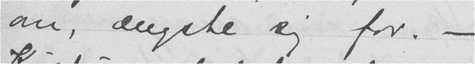om, engste sig for. -
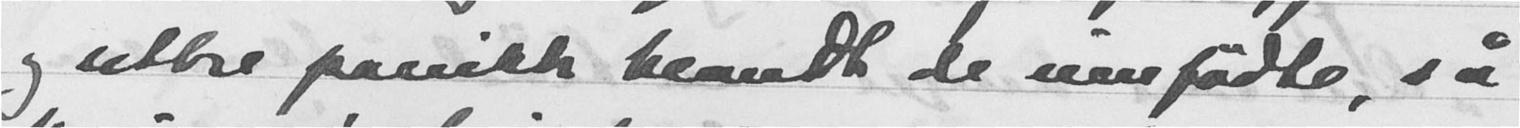og utbre panikk blandt de innfødte, så
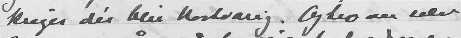krigen den blir kortvarig. Og tro om selv
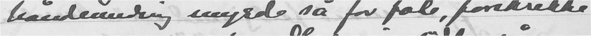håndvending myrde så for fote, forskrekke
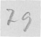79
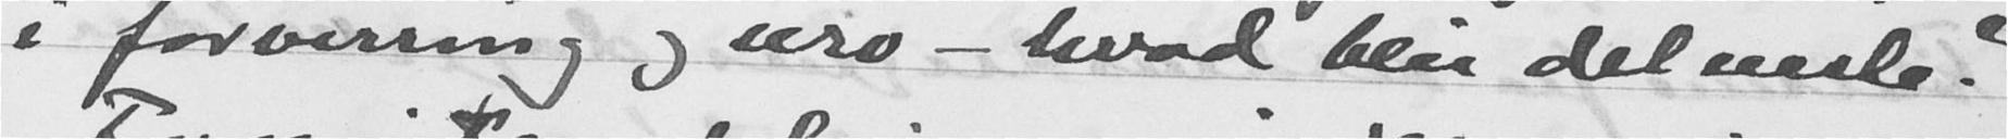i forvirring og uro - hvad blir det neste?
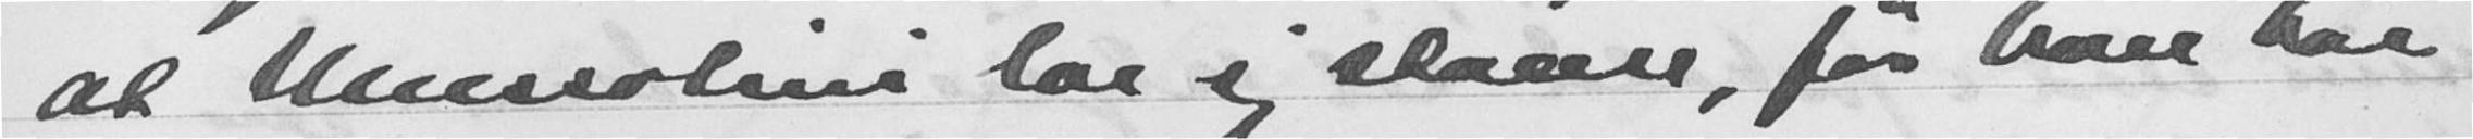at Mussolini lar sig stanse, før han har
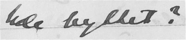hele byttet?
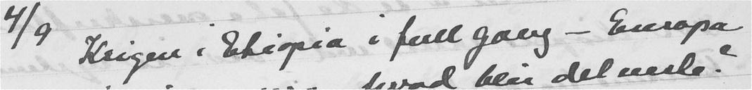4/9 Krigen i Etiopia i full gang - Europa
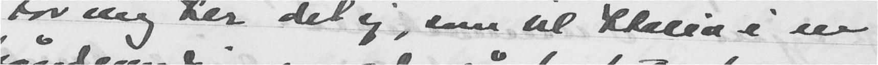For mig ter det sig, som vil Italia i en
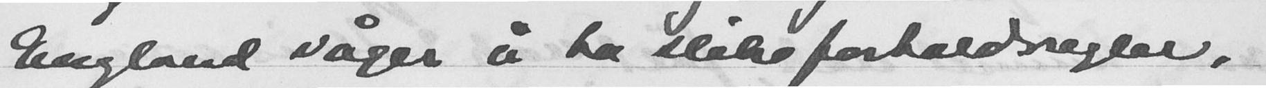England våger å ha slike forhåndsregler,
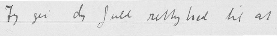Jeg gir dig fuld rettighed til at
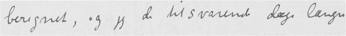beregnet, og jeg da tilsvarende dager længere
